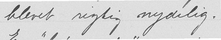blevet rigtig nydelig.
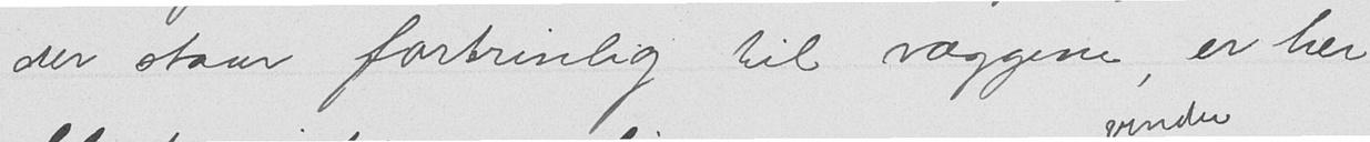der staar fortrinlig til væggene, er her
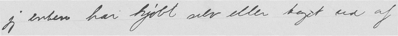jeg enten har kjøbt selv eller taget ud af
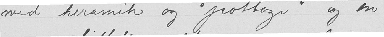med keramik og "pottage" og en
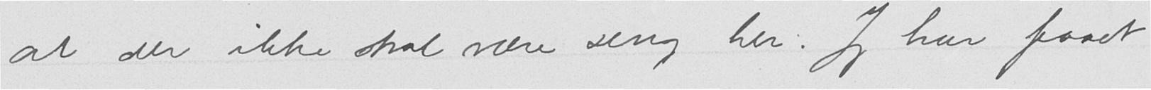at der ikke skal være seng her. Jeg har faaet
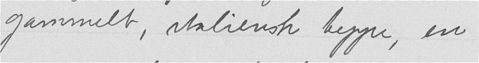gammelt, italiensk teppe, en
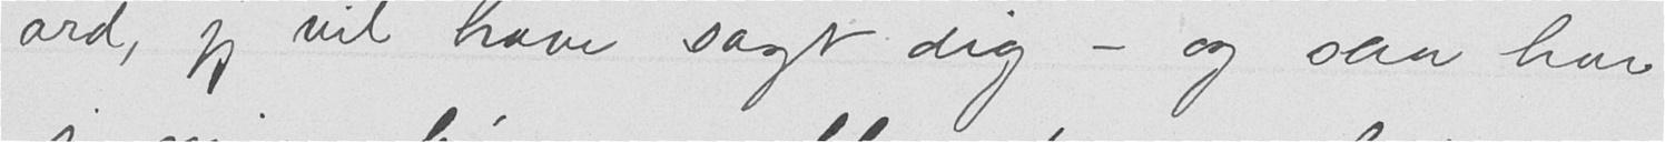ord, jeg vil have sagt dig - og saa har
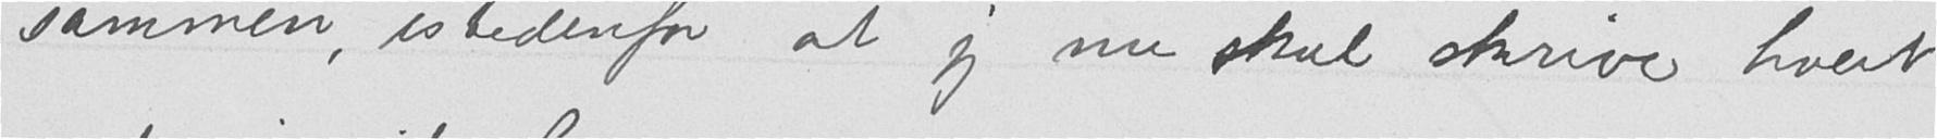sammen, istedenfor at jeg nu skal skrive hvert
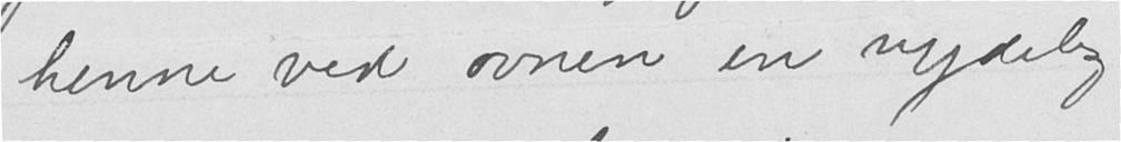henne ved ovnen en nydelig
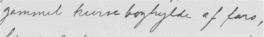gammel kurveboghylde af fars,
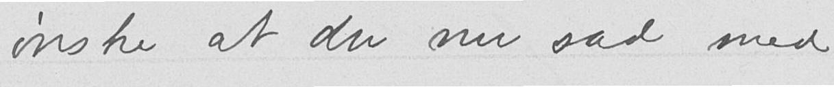ønske at du nu sad med
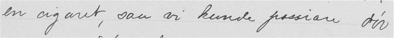en cigaret, saa vi kunde passiare tøv
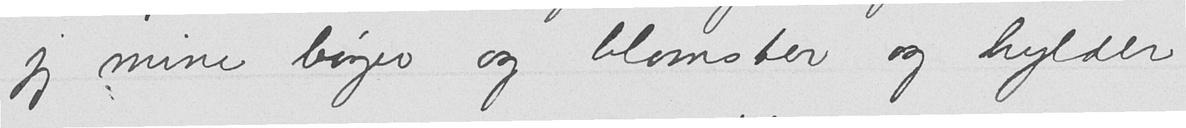jeg mine bøger og blomster og hylder
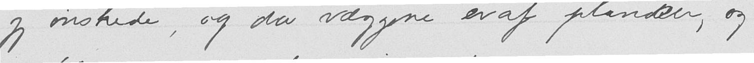jeg ønskede, og da væggene er af planker, og
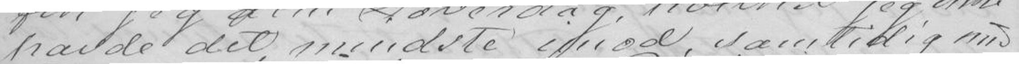havde det mindste imod, samtidig med
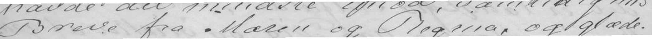Breve fra Maren og Regina, og glæde-
